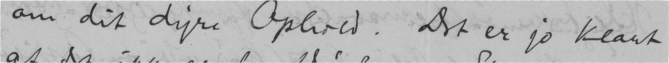om dit dyre Ophold. Det er jo klart
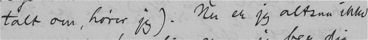talt om, hører jeg). Nu er jeg altsaa ikke
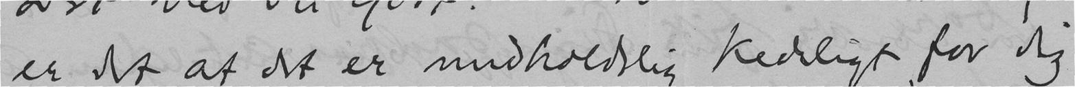er det at det er uudholdelig kedligt for dig
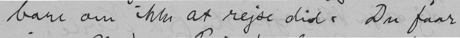bare om ikke at rejse did. Du faar
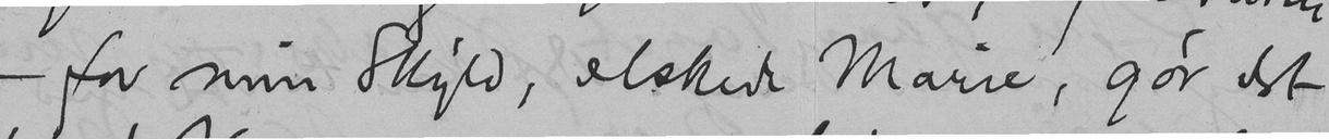- for min Skyld, elskede Marie, gør det
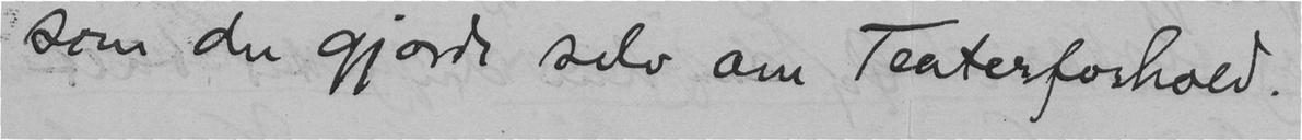som du gjorde selv om Teaterforhold.
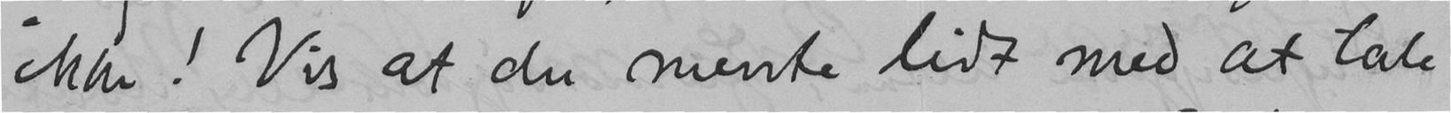ikke! Vis at du mente lidt med at tale
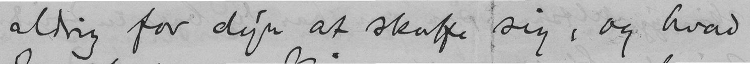aldrig for dyr at skaffe sig, og hvad
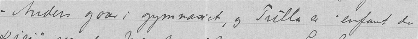- Anders gaar i gymnasiet, og Tulla er "enfant de
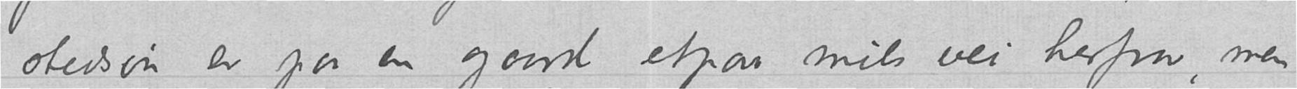stedsøn er paa en gaard etpar mils vei herfra, men
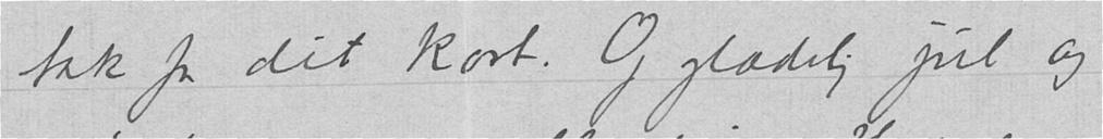tak for dit kort. Og glædelig jul og
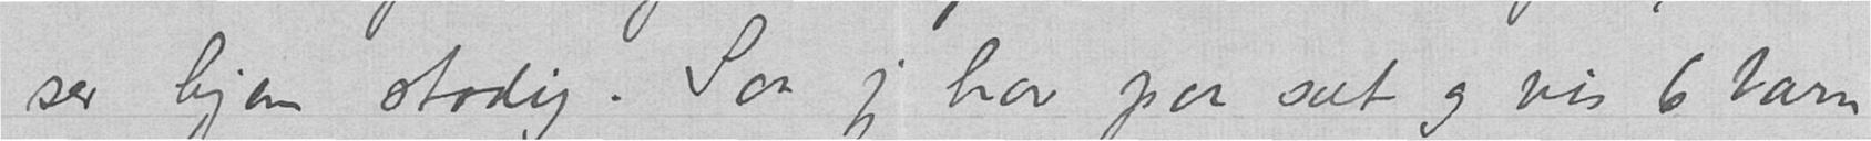ser hjem stadig. Saa jeg har paa sæt og vis 6 barn
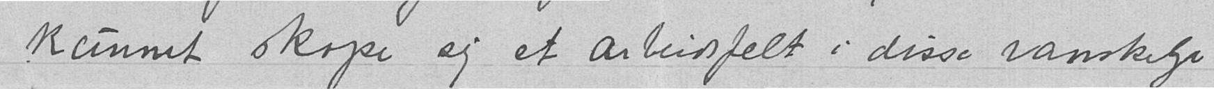kunnet skape sig et arbeidsfelt i disse vanskelige
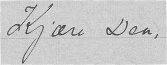Kjære Dea,
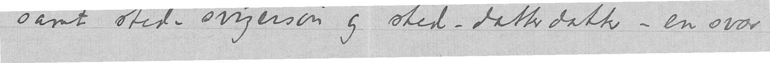samt sted-svigersøn og sted-datterdatter – en svær
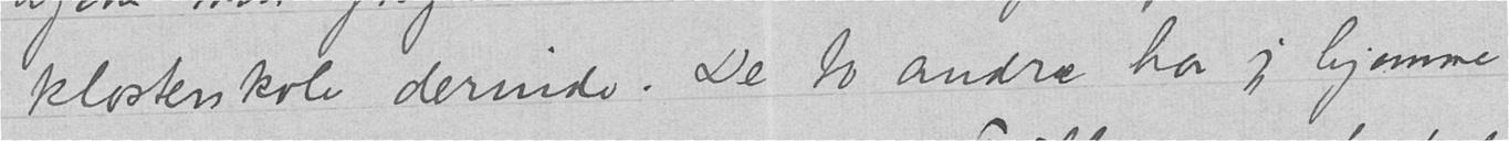klosterskole derinde. De to andre har jeg hjemme
